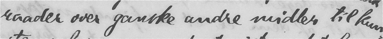raader over ganske andre midler til kun-
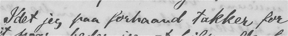Idet jeg paa forhaand takker for
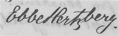Ebbe Hertzberg.
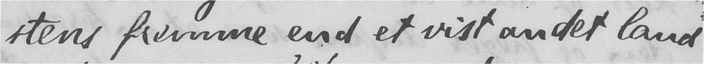stens fremme end et vist andet land
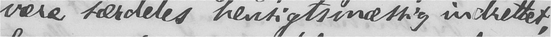være særdeles hensigtsmæssig indrettet,
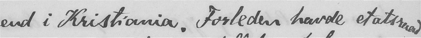end i Kristiania. Forleden havde etatsraad
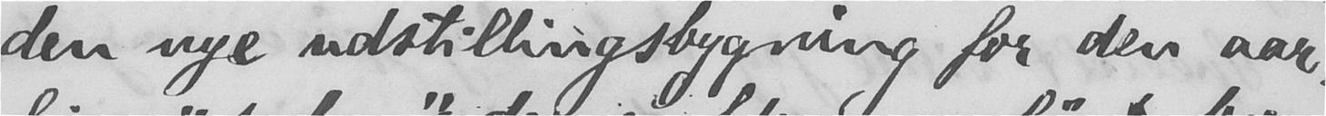den nye udstillingsbygning for den aar-
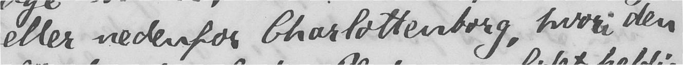eller nedenfor Charlottenborg, hvori den
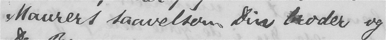Maurers saavelsom Din broder og
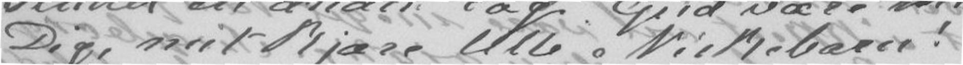Dig, mit kjære lille Niskebarn!
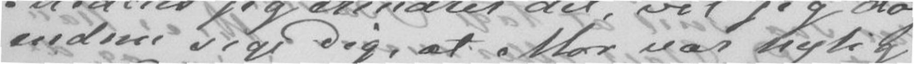endnu sige Dig, at Mor var nylig
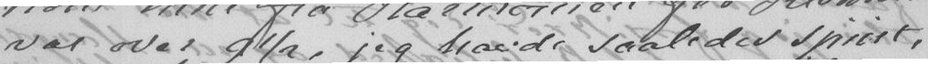var over 9½, jeg havde saaledes spiist,
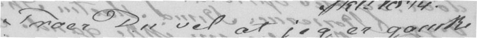Troer Du vel at jeg er ganske
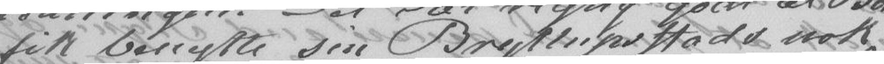fik benytte sin Bryllupsstads nok
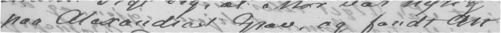paa Alexandras Grav, og fandt Alt
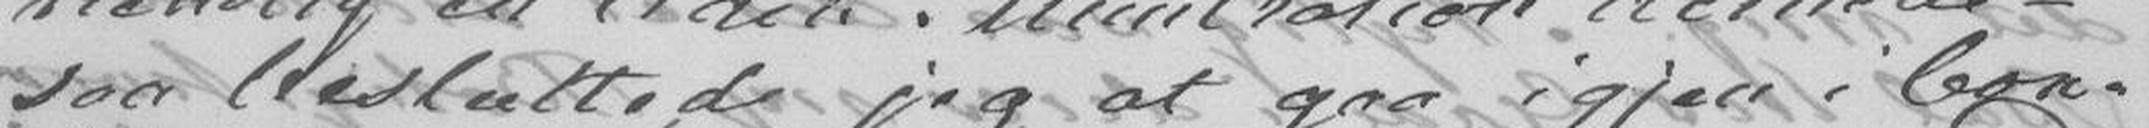saa besluttede jeg at gaa igjen i Con
